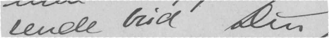sende bud Din
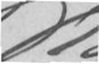B Bjørnson
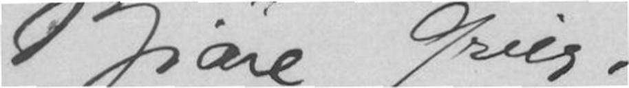Kjære Grieg!
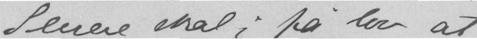Senere skal i få lov at
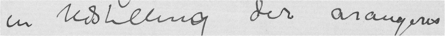en Udstilling der arangeres
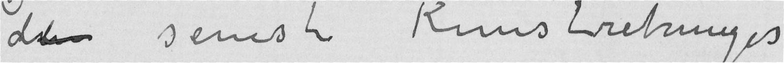de seneste Kunstretninger
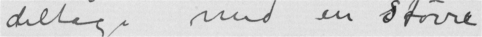deltage med en Sstørre
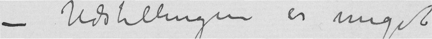– Udstillingen er meget
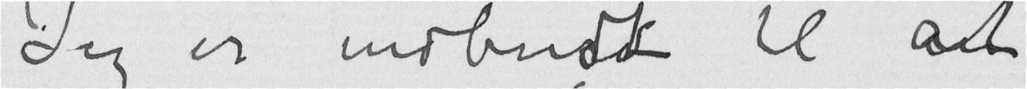Jeg er indbudt til at
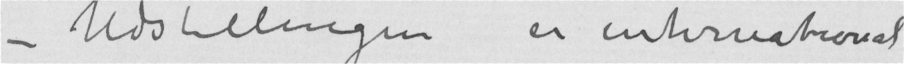– Udstillingen er international
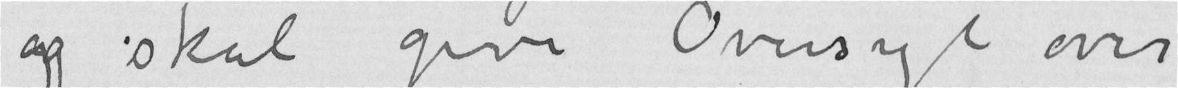og skal give Oversigt over
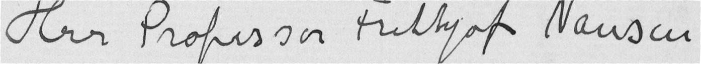Hrr Professor Fridthof Nansen
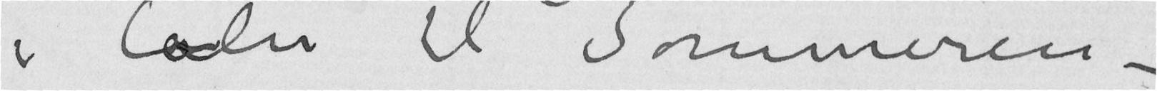i Cöln til Sommeren –
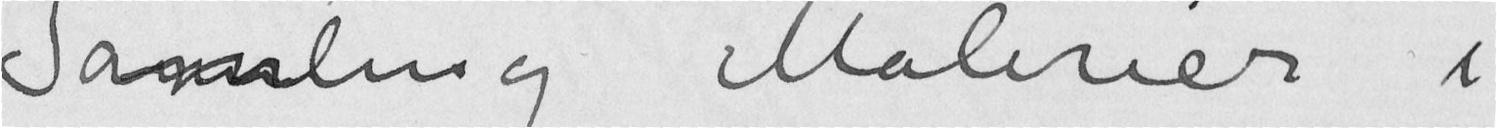Samling Malerier i
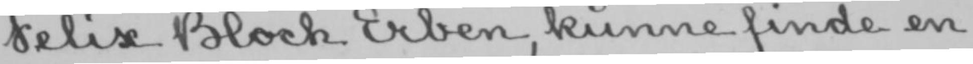Felix Bloch Erben, kunne finde en
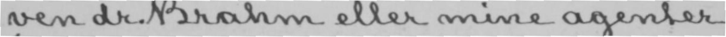ven dr. Brahm eller mine agenter,
